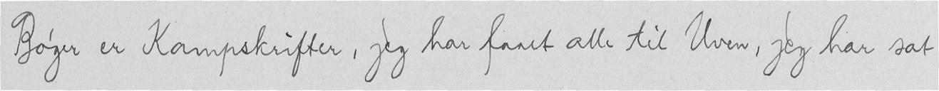Bøger er Kampskrifter, jeg har faaet alle til Uven, jeg har sat
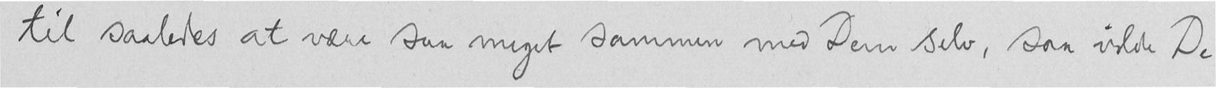til saaledes at være saa meget sammen med Dem selv, saa vilde De
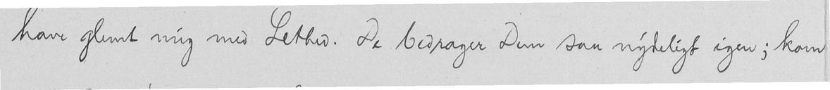have glemt mig med Lethed. De bedrager Dem saa nydeligt igen; kom
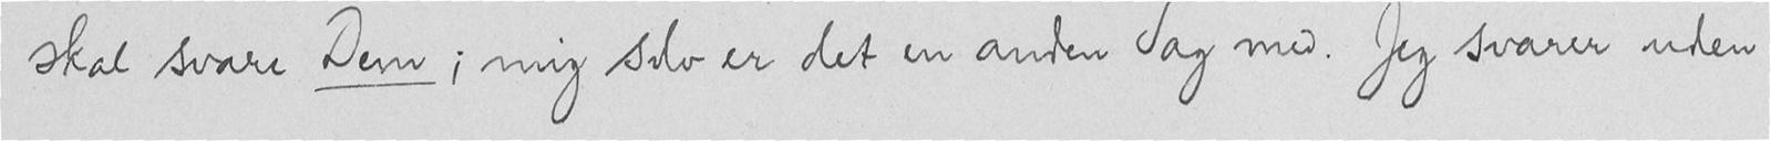skal svare Dem; mig selv er det en anden Sag med. Jeg svarer uden
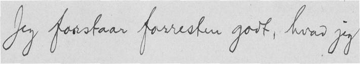Jeg forstaar forresten godt, hvad jeg
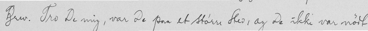Brev. Tro De mig, var De paa et større Sted, og De ikke var nødt
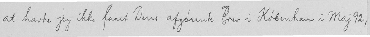at havde jeg ikke faaet Deres afgørende Brev i København i Maj 92,
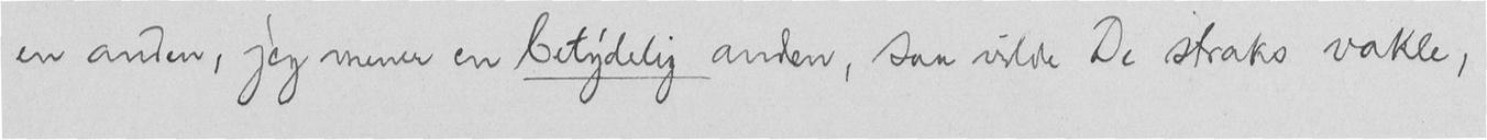en anden, jeg mener en betydelig anden, saa vilde De straks vakle,
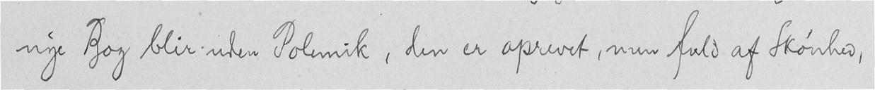nye Bog blir uden Polemik, den er oprevet, men fuld af Skønhed,
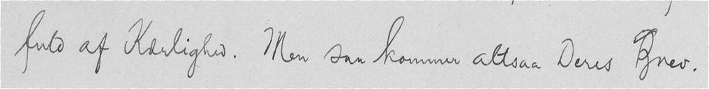fuld af Kærlighed. Men saa kommer altsaa Deres Brev.
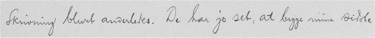Skrivning blevet anderledes. De har jo set, at begge mine sidste
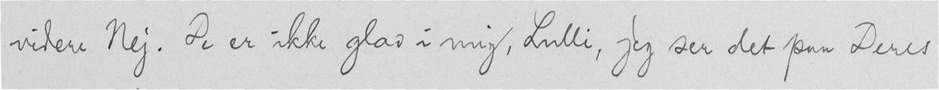videre Nej. De er ikke glad i mig, Lulli, jeg ser det paa Deres
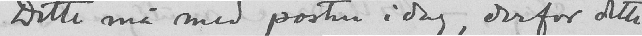Dette må med posten i dag, derfor dette
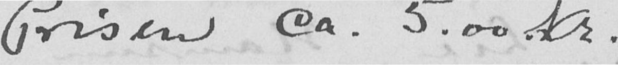Prisen ca. 5.00. Kr.
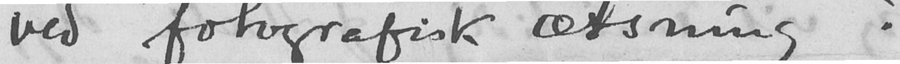ved fotografisk ætsning?
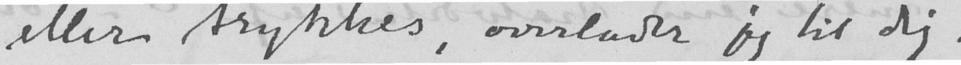eller trykkes, overlader jeg til dig.
